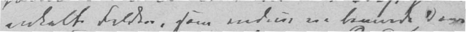enkelte Feldte, som endnu ere kunde deres
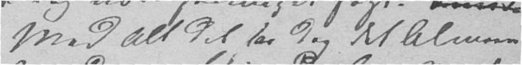Med Alt det har dog det Almeen-
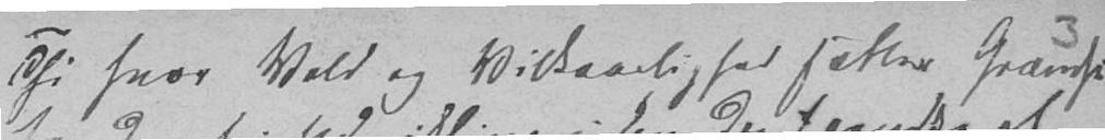Thi hvor Vold og Vilkaarlighed sætter Grændse
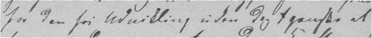for den fri Udvikling uden dog ganske at
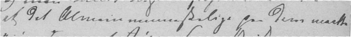at det Almeenmenneskelige paa dem maatte
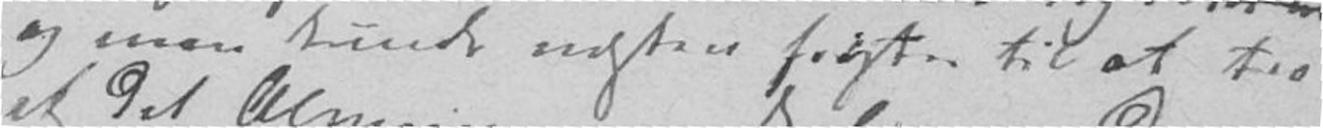og man kunde næsten friste til at tro
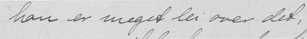han er meget lei over det,
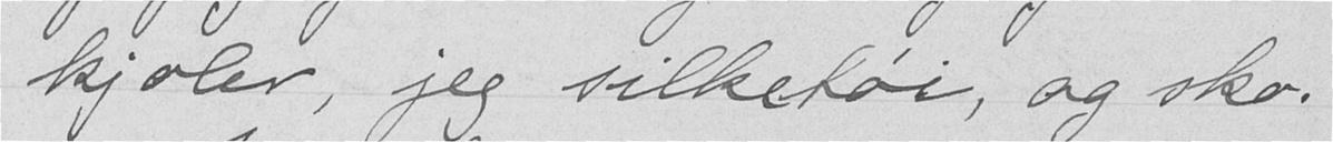kjoler, jeg silketøi, og sko.
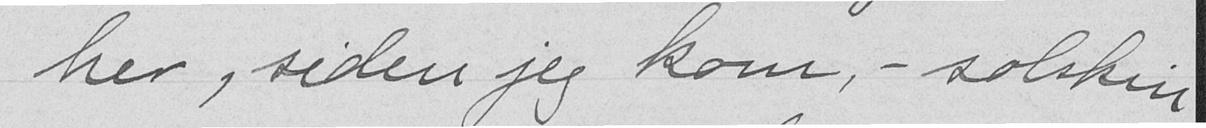her, siden jeg kom, - solskin
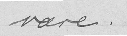være.
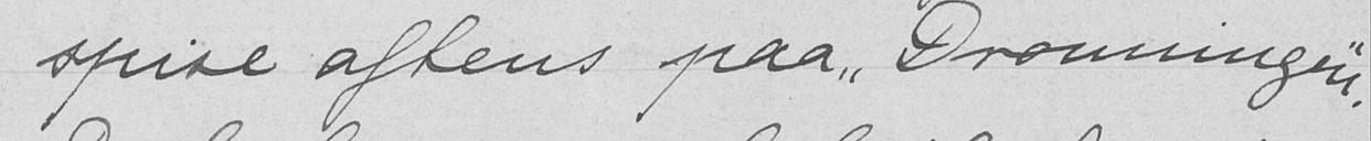spise aftens paa Dronningen.
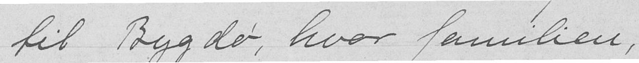til Bygdø, hvor famlien,
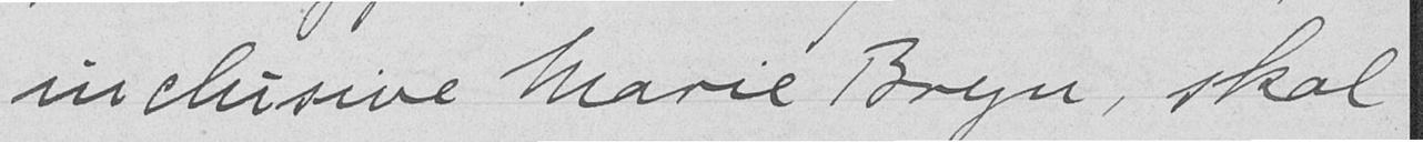inclusive Marie Bryn, skal
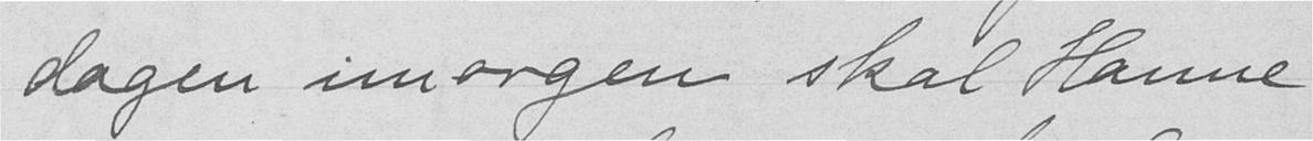dagen imorgen skal Hanne
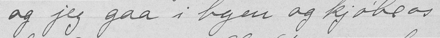og jeg gaa i byen og kjøbe os
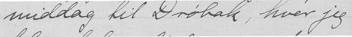middag til Drøbak, hvor jeg
